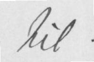til.
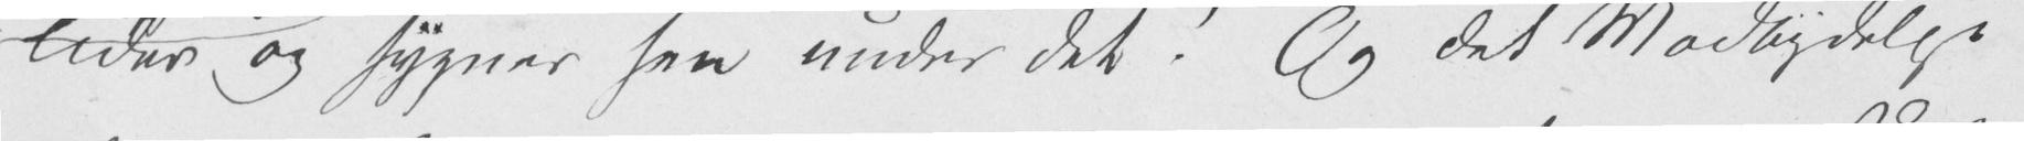lider og sygner hen under det! Og det Modbydelige
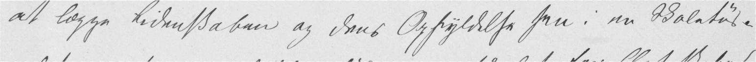at lægge Lidenskaben og dens Opfyldelse hen i en Skoletøs.
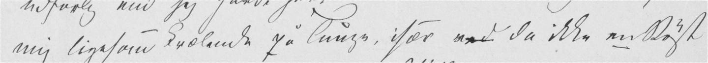mig ligesom kvælende på Tunge, især ved da ikke en Røst
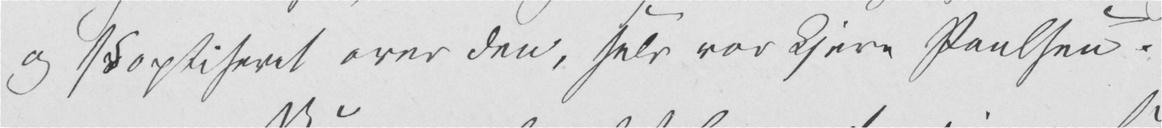og sopliseret over den, selv vor kjere Paulsen.
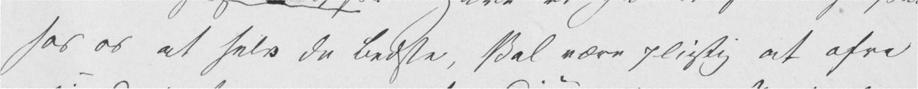hos os at selv de Bedste, skal være pligtig at ofre
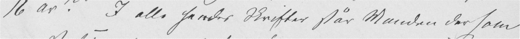16 År? I alle hendes Skrifter står Manden der som
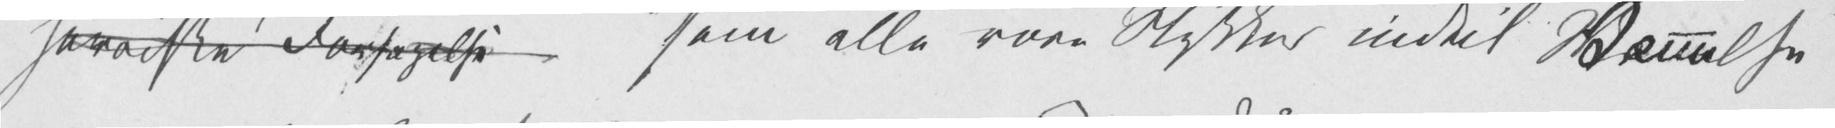soraiske Forsagelse som alle vore Stykker indtil Mænelse
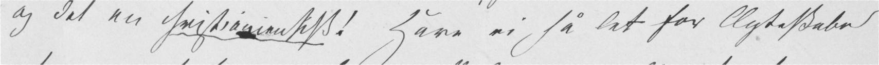og det en christianiansisk! Have vi så let for Ægteskaber
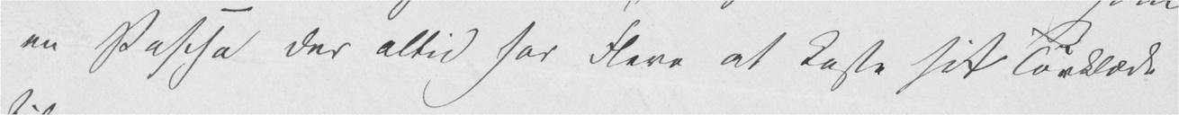en Pascha der altid har Flere at kaste sit Tørklæde
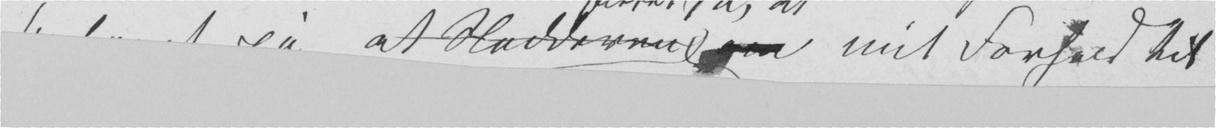et på at Sladderen om mit Forhold til
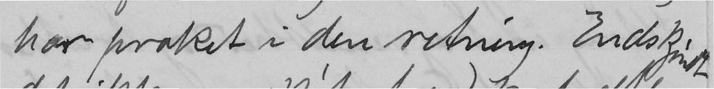har præket i den retning. Endskjønt
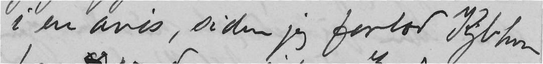i en avis, siden jeg forlod Kjbhvn
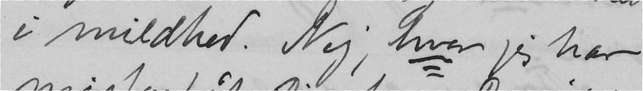i mildhed. Nej, hvor jeg har
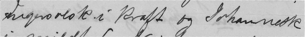Ingersolsk i kraft og johannesk
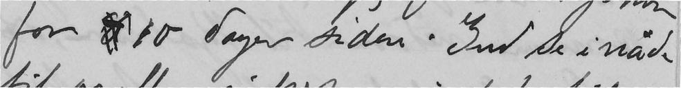for 8 10 dager siden. Gud se i nåde
